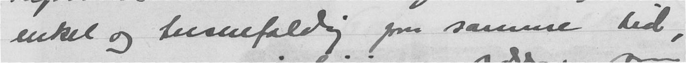enkel og tusenfoldig paa samme tid,
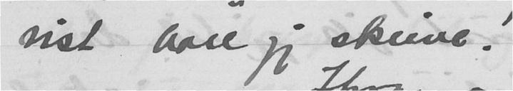vist bare jeg skrive!
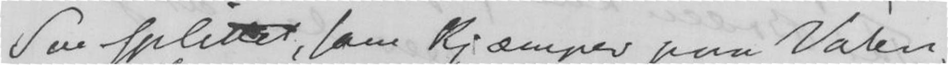Saa spittet, som Kjæmper paa Valen,
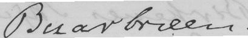Buarbreen.
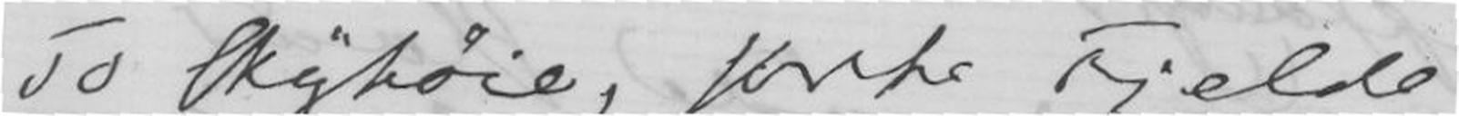To Skyhøie, korte Fjelde
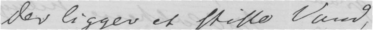der ligger et stille Vand,
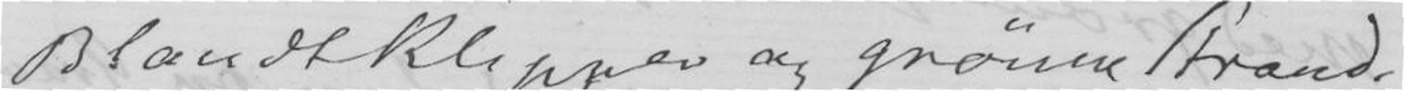Blandt klipper og grønne Strand.
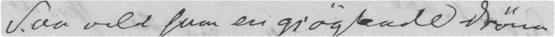Saa vild paa en gjøglende drøm
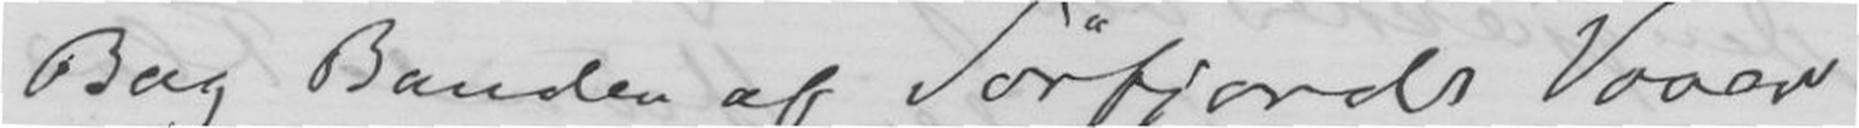Bag Banden af Sørfjord Vover
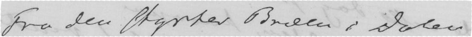Fra den Styrter Bræen i Dalen
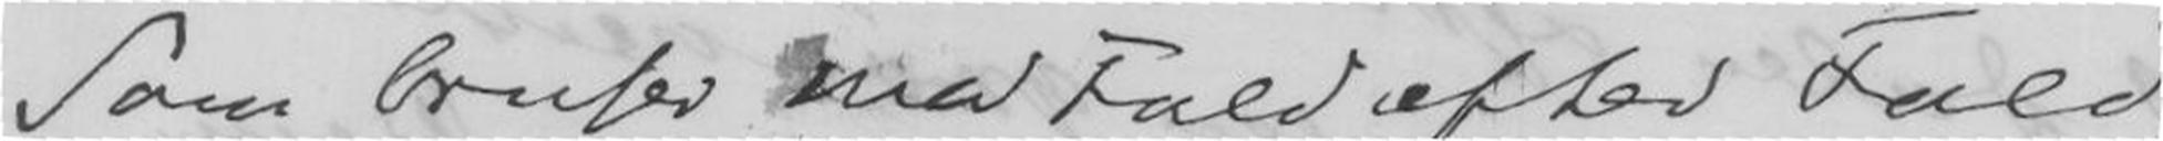Som bruser ned Fald efter Fald,
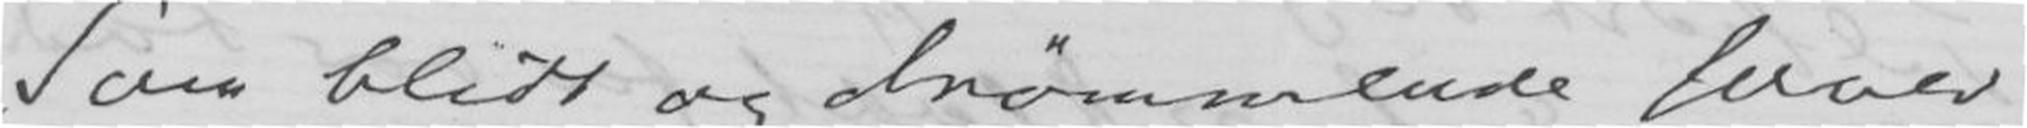Som blidt og drømmende svaer
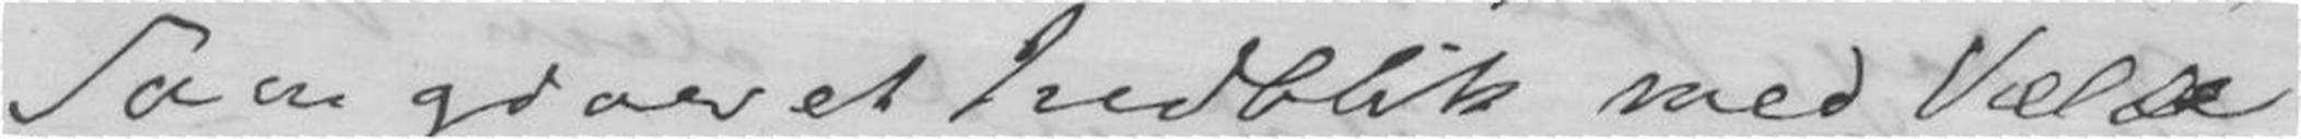Som giver et Indblik med Vælde
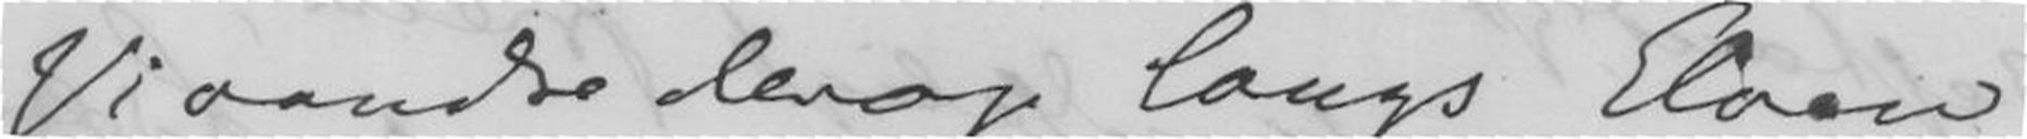Vi vandre derop langs Elven
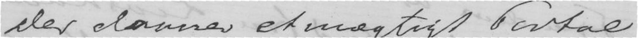Der danner et mægtigt Portal,
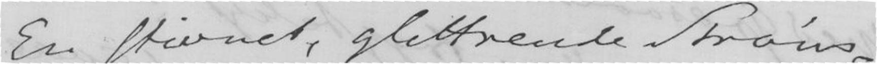En stivnet, glittrende Strøm,
